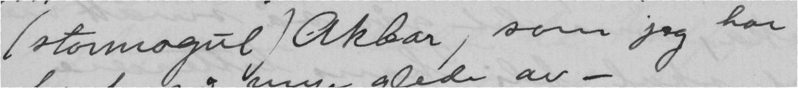(stormogul) Akbar, som jeg har
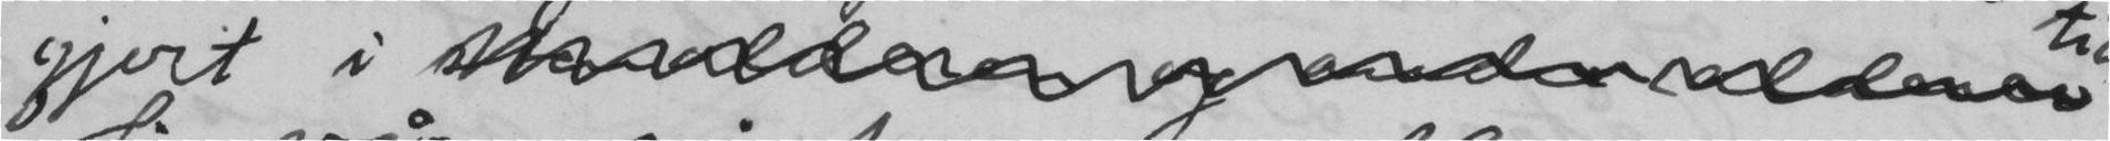gjort i
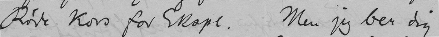Røde Kors for Ekspl. Men jeg ber dig
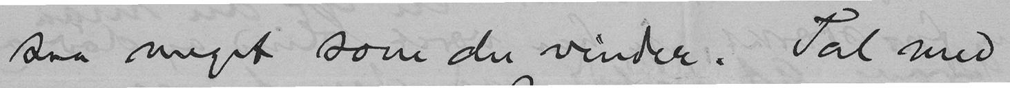saa meget som du vinder. Tal med
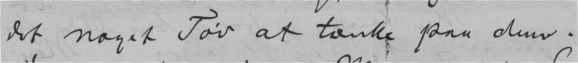det noget Tøv at tænke paa dem.
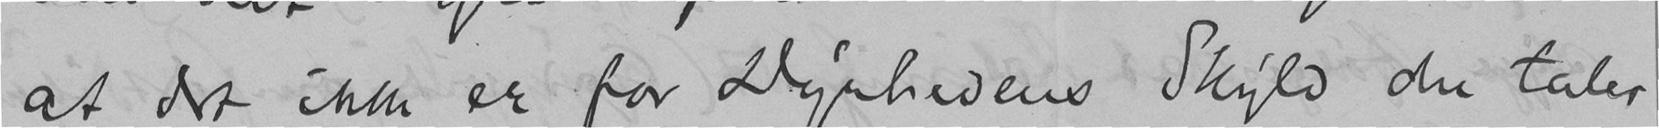at det ikke er for Dyrhedens Skyld du taler
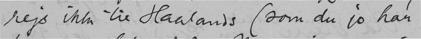rejs ikke til Haalands (som du jo har
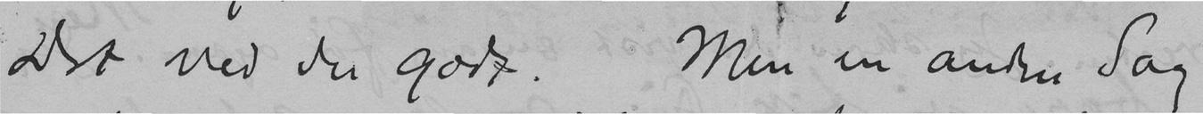Det ved du godt. Men en anden Sag
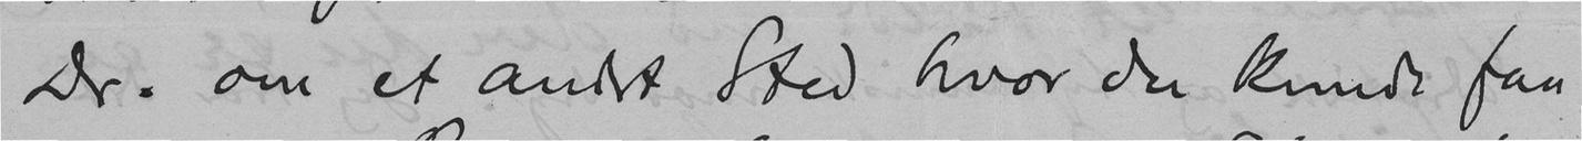Dr. om et andet Sted hvor du kunde faa
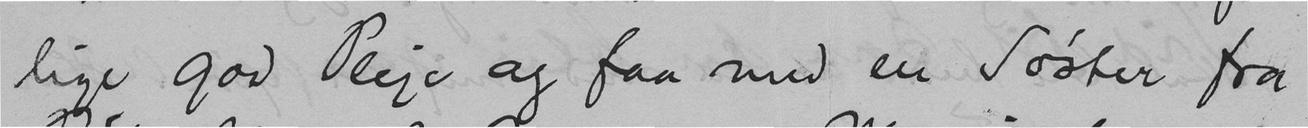lige god Pleje og faa med en Søster fra
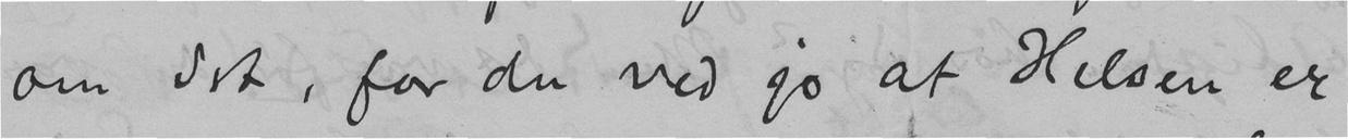om det, for du ved jo at Helsen er
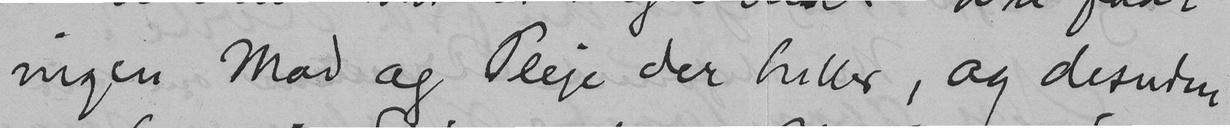ingen Mad og Pleje der heller, og desuden
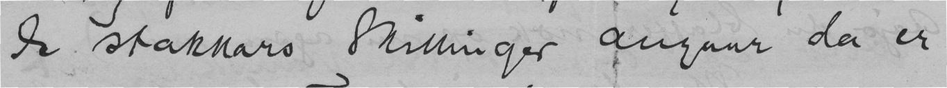de stakkars Skillinger angaar da er
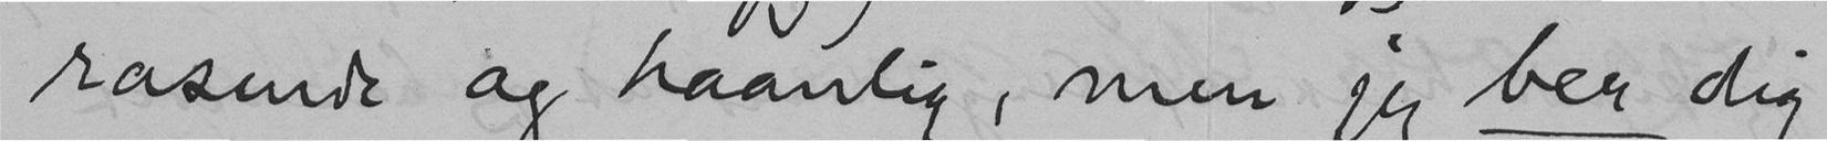rasende og haanlig, men jeg ber dig
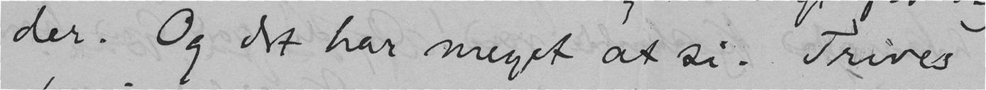der. Og det har meget at si. Trives
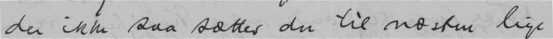du ikke saa sætter du til næsten lige
